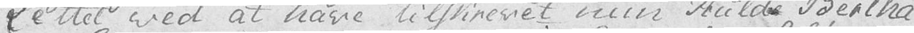Lettet ved at have tilskrevet min Hulde Bertha
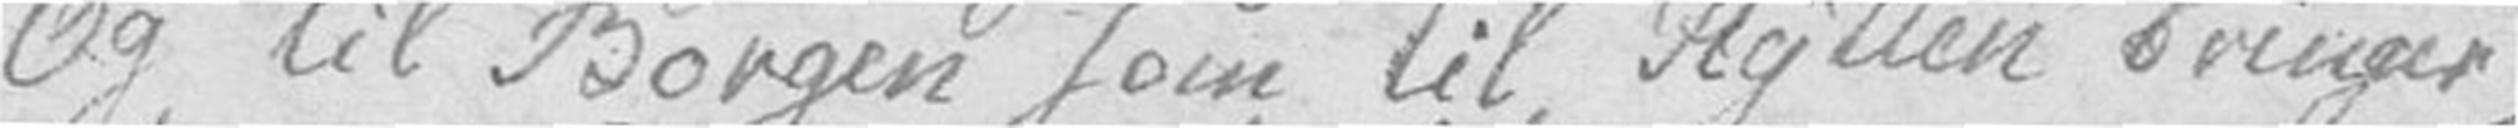Og til Borgen som til Hytten bringer
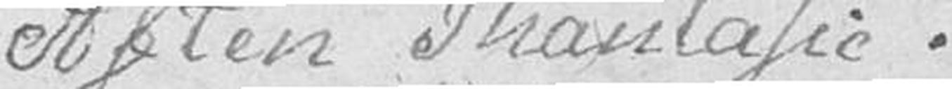Aften Phantasie.
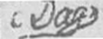i Dag
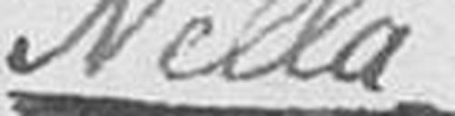Nella
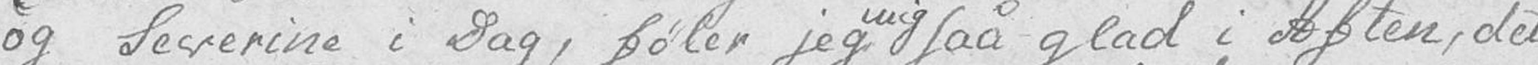og Severine i Dag, føler jeg mig saa glad i Aften, det
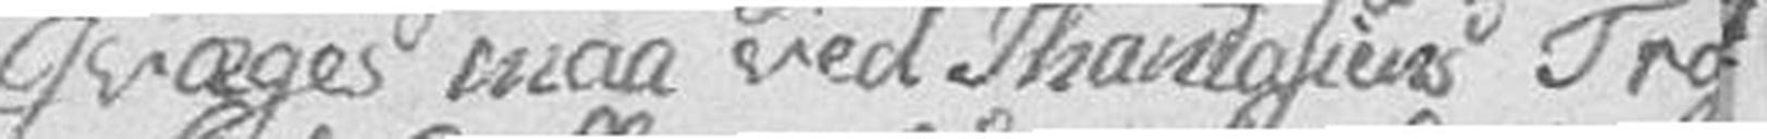qvæges man ved Phantasiens Tro
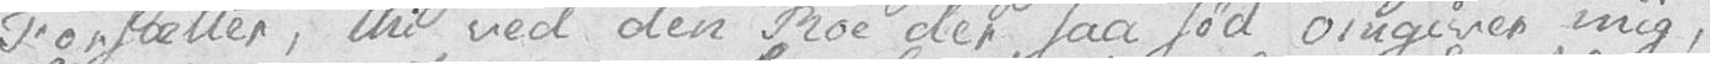Forsætter, thi ved den Roe der saa sød omgiver mig,
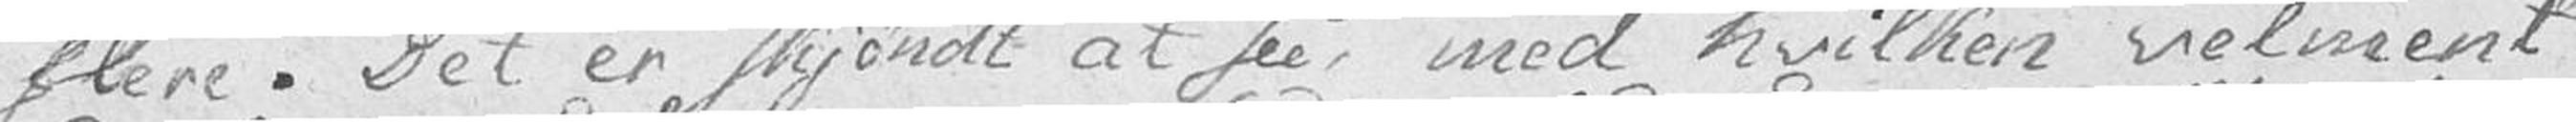flere. Det er skjøndt at see, med hvilken velment
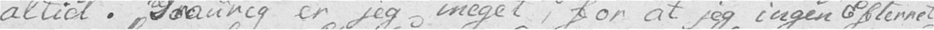altid. Traurig er jeg meget, for at jeg ingen Efterret
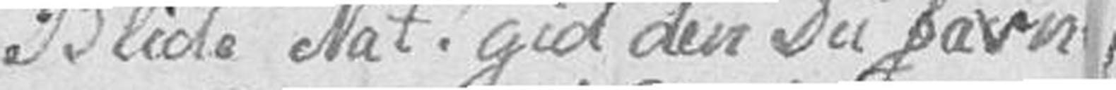Blide Nat! gid den Du favn
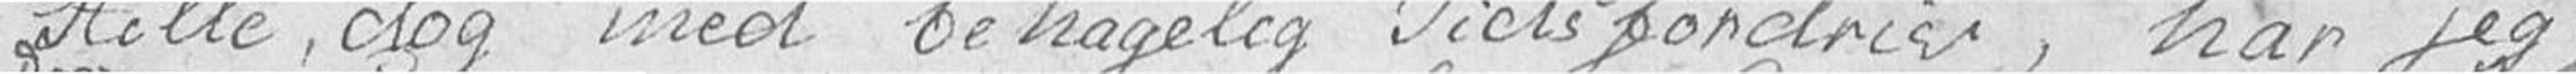Stille, dog med behagelig Tidsfordriv, har jeg
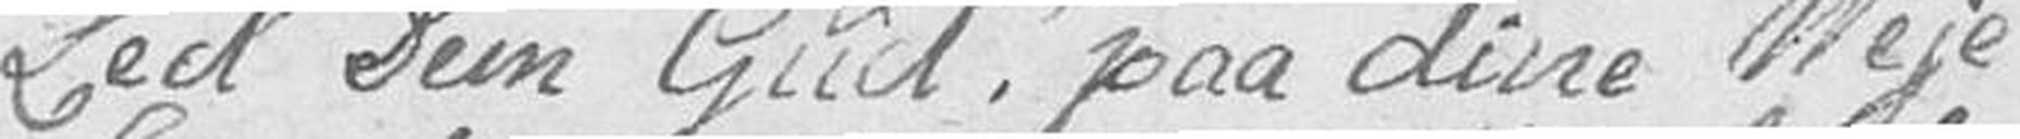Led Dem Gud! paa dine Veje
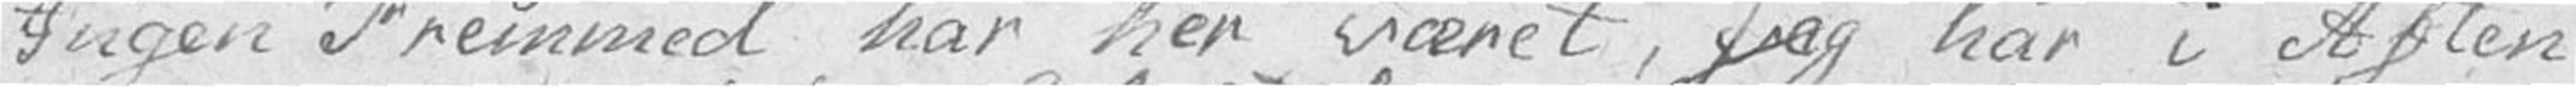Ingen Fremmed har her været, jeg har i Aften
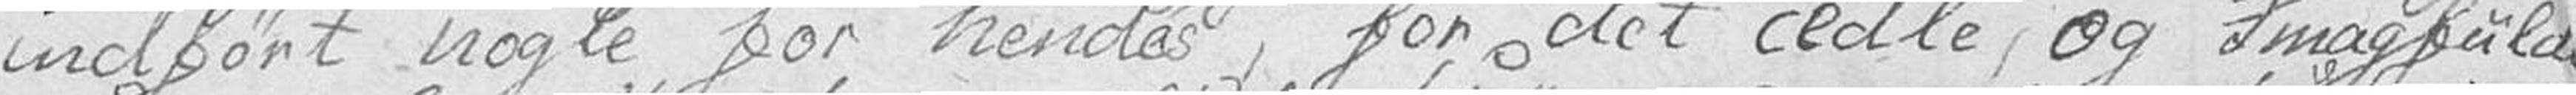indført nogle for hendes, for det ædle, og Smagfulde
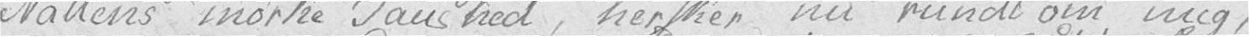Nattens mørke Taushed, hersker nu rundt om mig,
